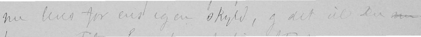nu leves for ens egen skyld, og det vil Du nu
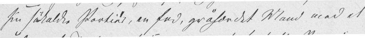sin såkaldte Portier, en fed, gråhærdet Mand med et
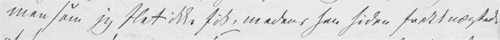men som jeg slet ikke fik, medens han siden frækt nægtede
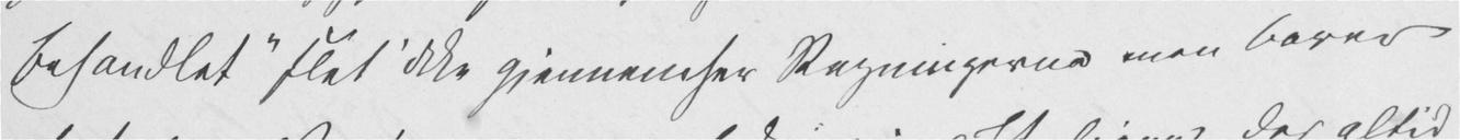behandlet" slet ikke gjennemser Regningerne men barer
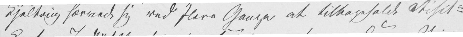Kjeltring hævnede sig ved flere Gange at tilbageholde Visit-
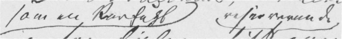som en Porfalle reserverende
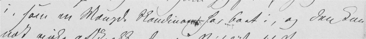i, som en Mængde Skandinaver har boet i, og den kan
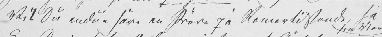Vil Du endnu have en Prøve på Romertilstande, så
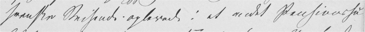svenske Reisende oplevede i et andet Pensionshu
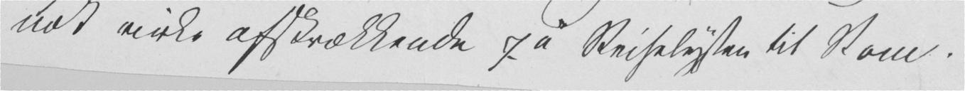nok virke afskrækkende på Reiselysten til Rom.
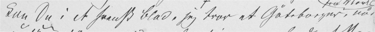kan Du i et svensk Blad, jeg tror et Gøteborger, når
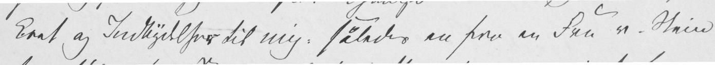kort og Indbydelser til mig. således en fra en Fru v. Stein
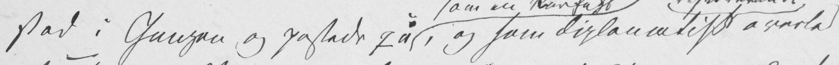stod i Gangen og passede på, og som diplomatisk overlod
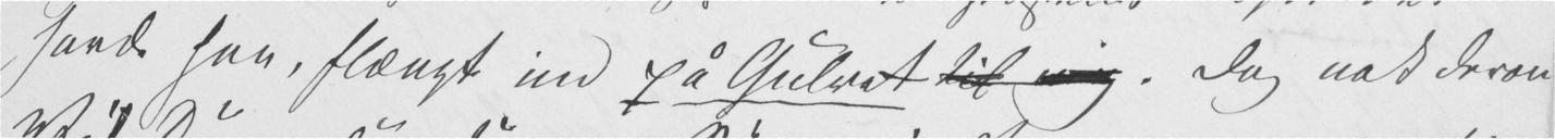havde han, slængt ind på Gulvet til mig. Dog nok derom
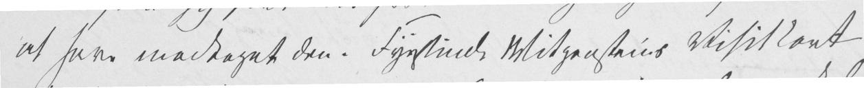at have modtaget den. Fyrstinde Witgensteins Visitkort
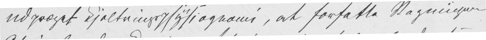udpræget Kjeltringsphysiognomi, at forfatte Regningen
